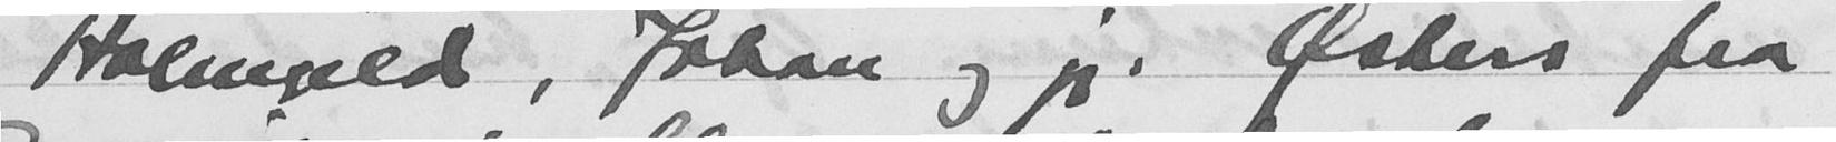Holmgild, Johan og jeg. Østers fra
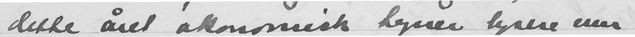dette året økonomisk synes lysere enn
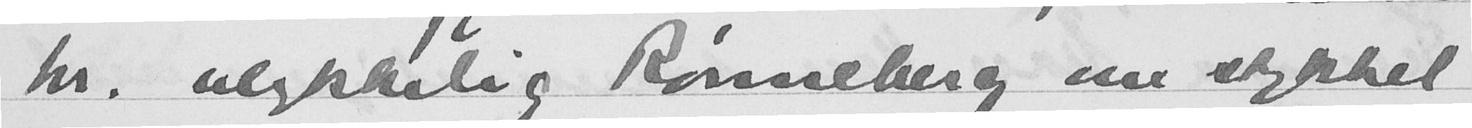hr. ulykkelig Rønneberg om stykket
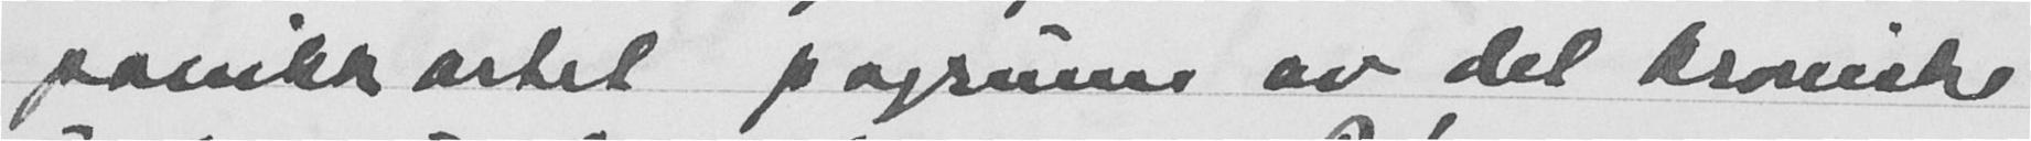panikkartet pågrunn av det kroniske
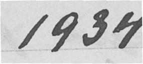1934
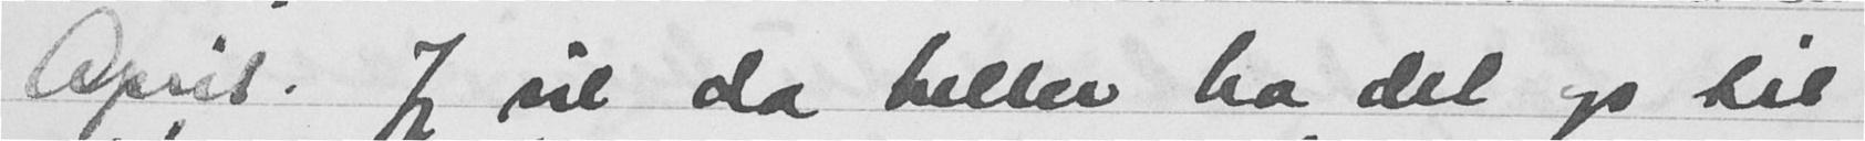April. Jeg vil da heller ha det op til
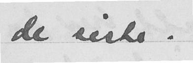de siste. -
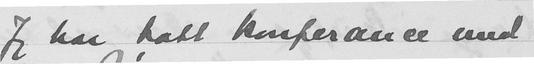Jeg har hatt konferance med
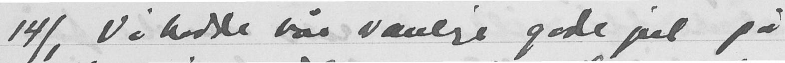14/1 Vi hadde vår vanlige gode prat på
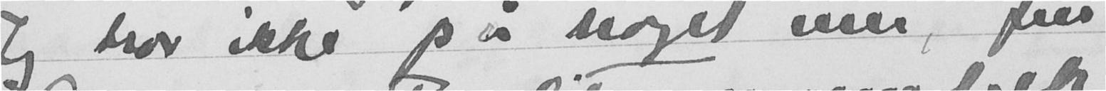Jeg tror ikke på noget mer, fru
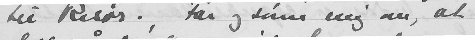til Risør. Far og sønn enig om, at
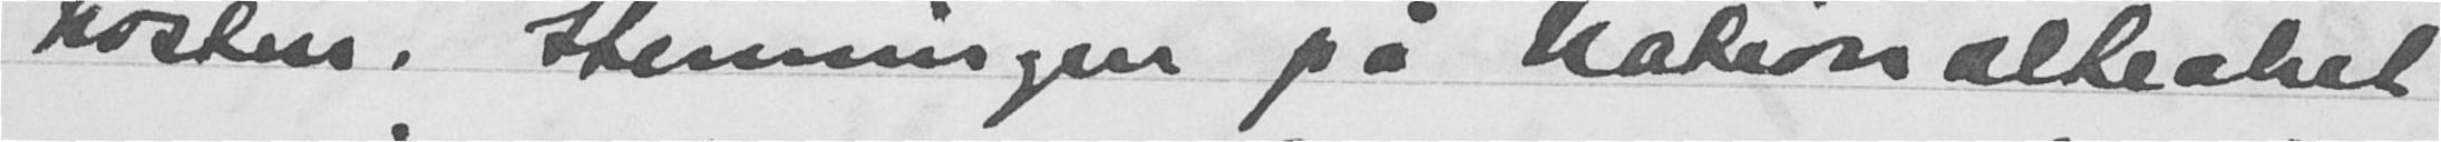høsten. Stemningen på Nationaltheatret
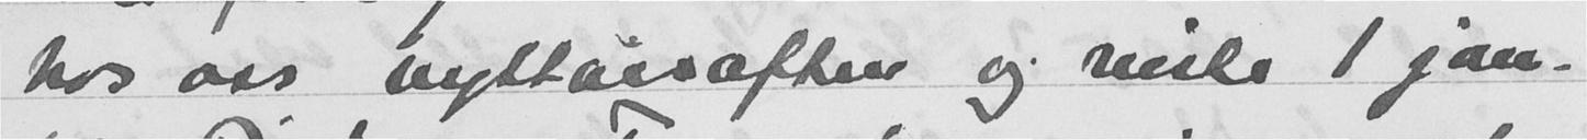hos oss nyttårsaften og reiste 1 jan.
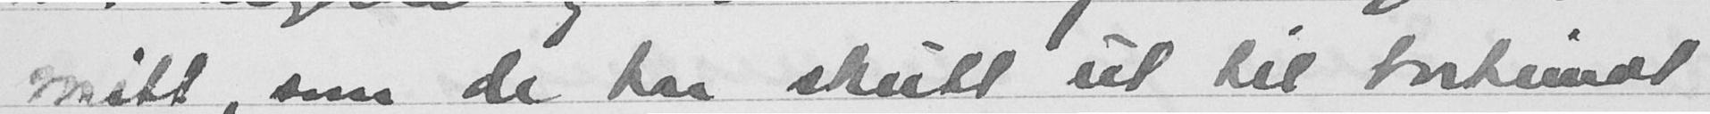mitt, som de har skutt ut til bortimot
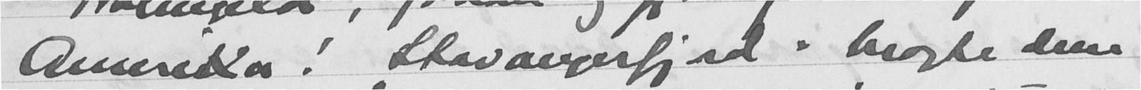Amerika! Stavangerfjord bragte dem
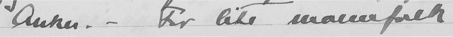Anker. - For lite mannfolk
